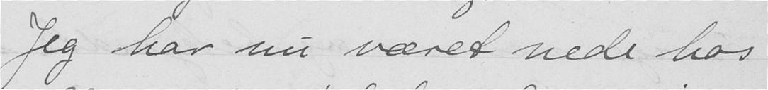Jeg har nu været nede hos
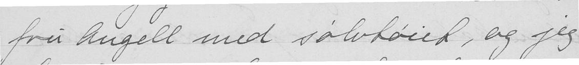fru Angell med sølvtøiet, og jeg
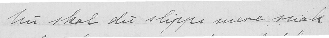Nu skal du slippe mere snak
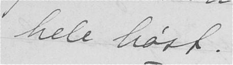hele høst.
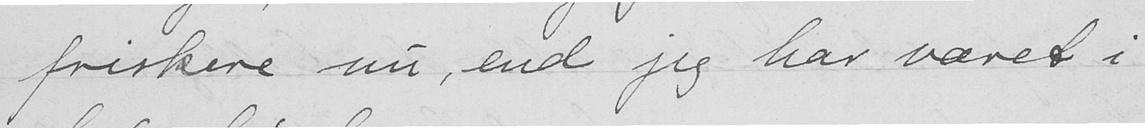friskere nu, end jeg har været i
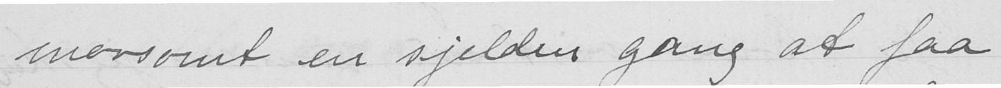morsomt en sjelden gang at faa
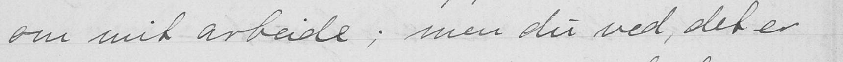om mit arbeide; men du ved, det er
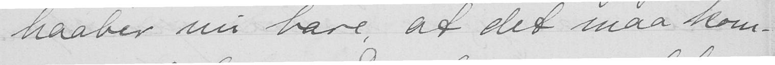haaber nu bare, at det maa kom-
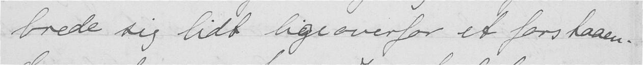brede sig lidt ligeoverfor et forstaaen-
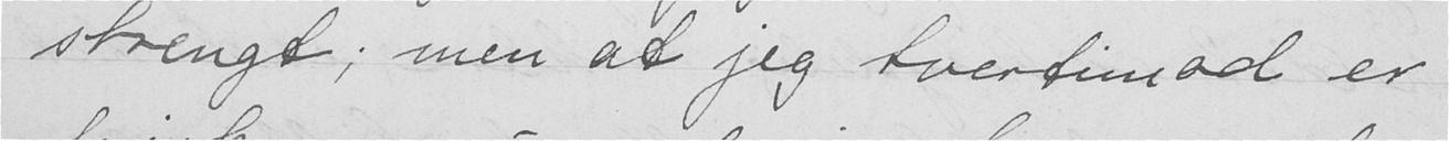strengt; men at jeg tvertimod er
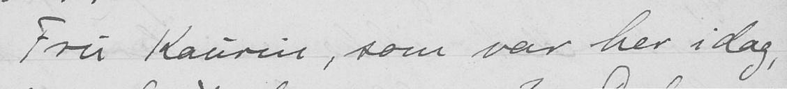Fru Kaurin, som var her idag
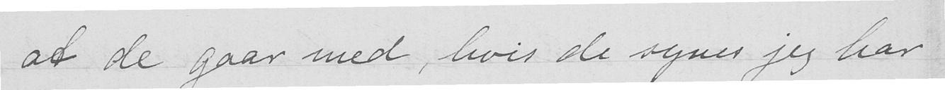at de gaar med, hvis de synes jeg har
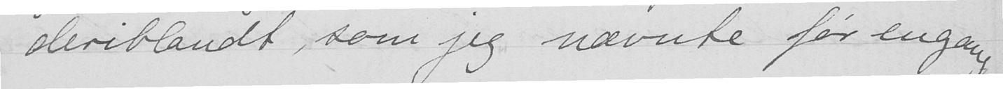deriblandt, som jeg nævnte før engang,
